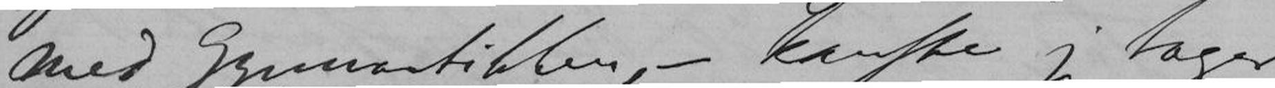med Gymnastikken, - kanske jeg tager
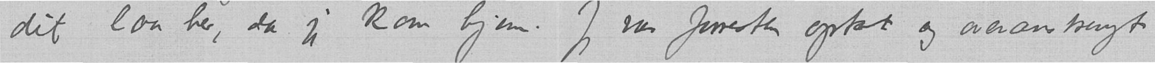dit laa her, da jeg kom hjem. Jeg var forresten optat og overanstrengt
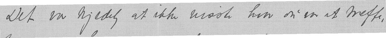Det var kjedelig at ikke visste, hvor du var at treffe,
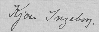Kjære Ingeborg,
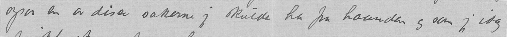ogsaa en av disse sakerne jeg skulde ha fra haanden og som jeg idag
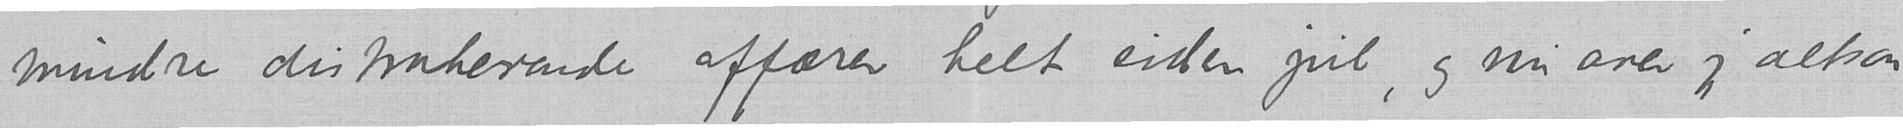mindre distraherende affærer helt siden jul, og nu aner jeg altsaa
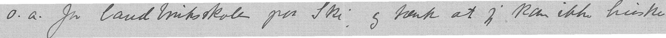o.a. for landbruksskolen paa Ski, og tænk at jeg kan ikke huske
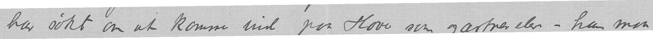har søkt om at komme ind paa Have som gartnerelev – han maa
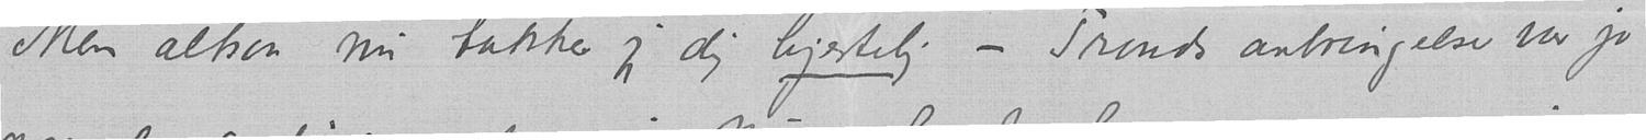Men altsaa nu takker jeg dig hjertelig – Tronds anbringelse var jo
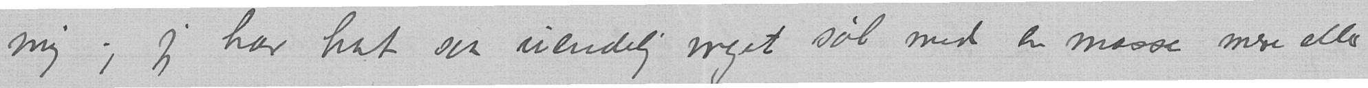ning ; jeg har hat saa uendelig meget søl med en masse mere eller
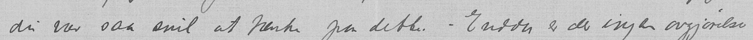du var saa snil at tænke paa dette. – Endda er der ingen avgjørelse
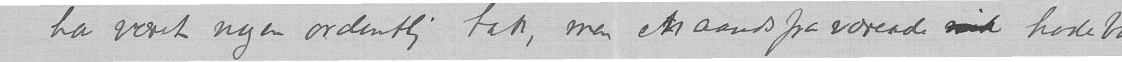ha været nogen ordentlig tak, men en aandsfraværende vink hodebøi-
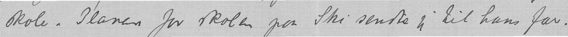skole . Planen for skolen paa Ski sendte jeg til hans far.
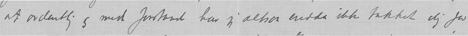at ordentlig og med forstand har jeg altsaa endda ikke takket dig for
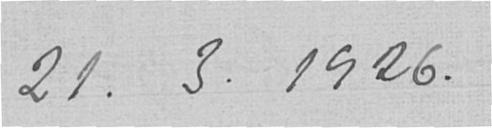21.3.1926.
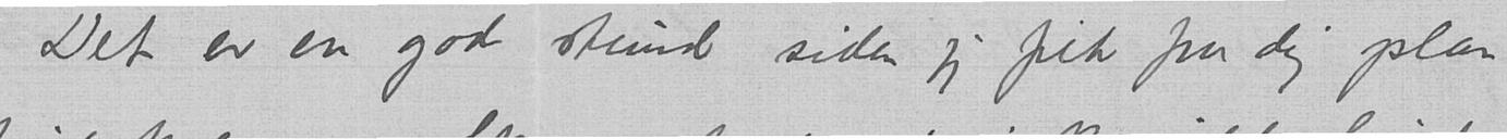Det er en god stund siden jeg fik fra dig plan
